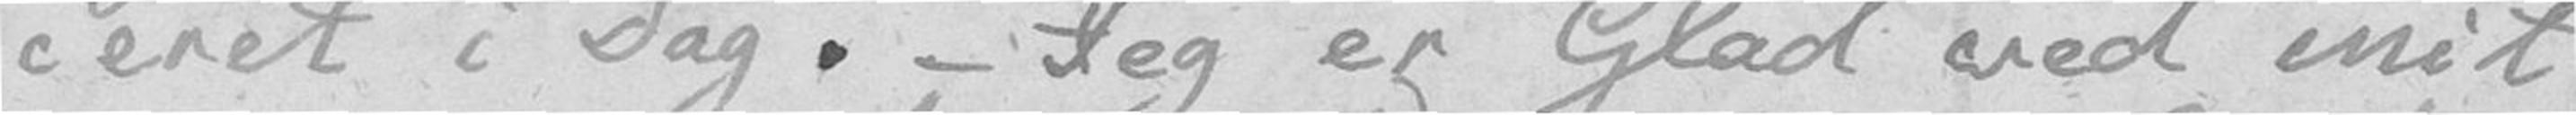ceret i Dag. – Jeg er Glad ved mit
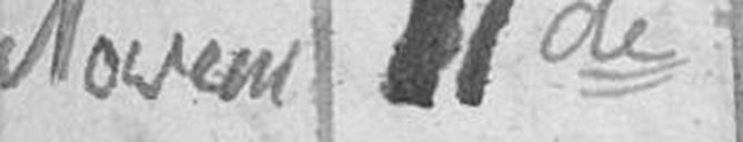Novem 11de
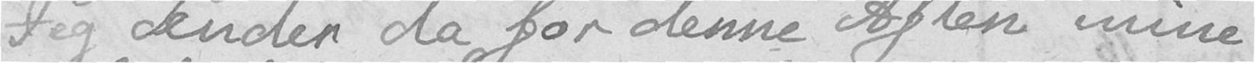Jeg ender da for denne Aften mine
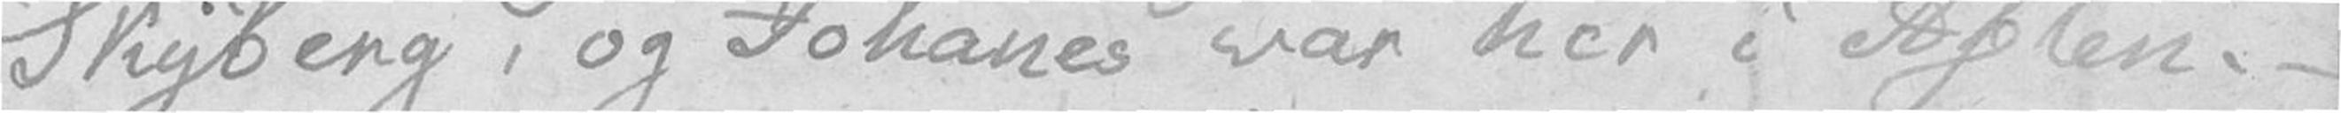Skyberg, og Johanes var her i Aften. –
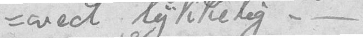-ved lykkelig – –
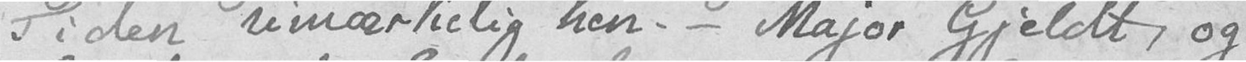Tiden umærkelig hen. – Major Gjeldt, og
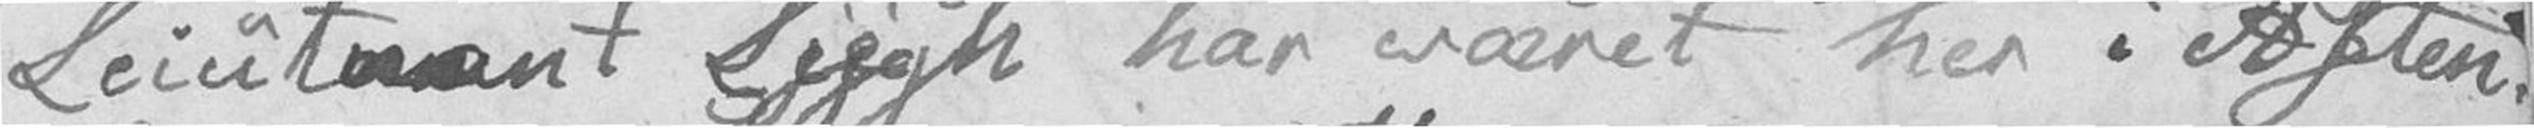Leiutnant Leigh har været her i Aften.
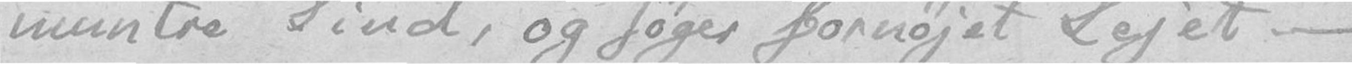muntre Sind, og søger fornøjet Lejet –
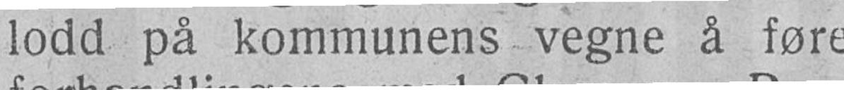lodd pà kommunens vegne å føre
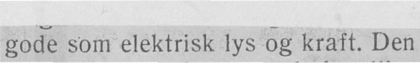gode som elektrisk lys og kraft. Den
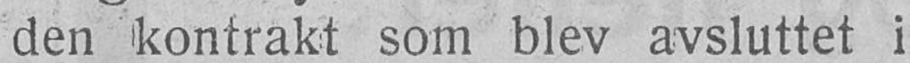den kontrakt som blev avsluttet i
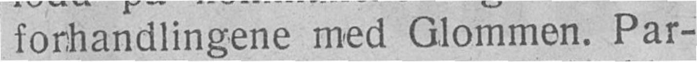forhandlingene med Glommen. Par-
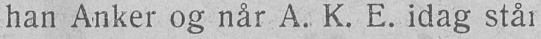han Anker og når A. K. E. idag står
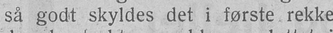så godt skyldes det i første rekke
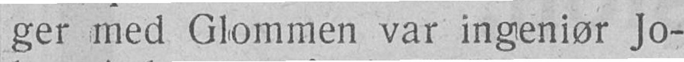ger med Glommen var ingeniør Jo-
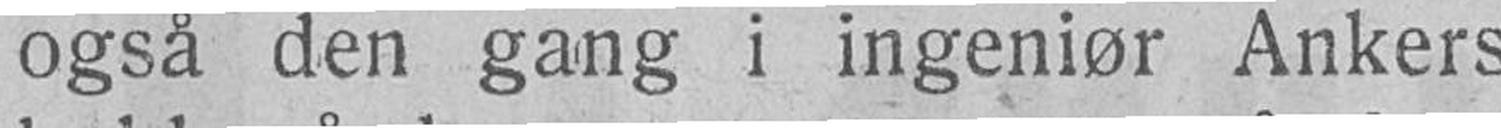også den gang i ingeniør Ankers
